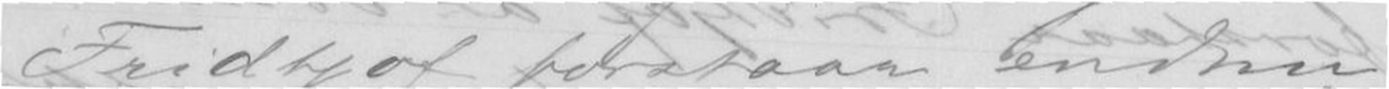Fridtjof forstaar endnu
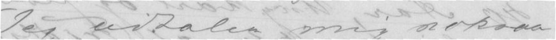Jeg udtaler mig noksaa
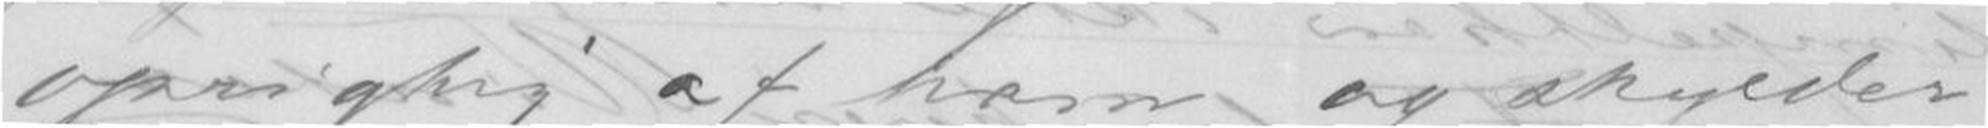oprigtig af ham og skylder
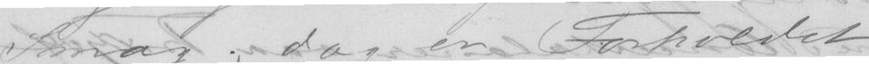Smag; dog er Forholdet
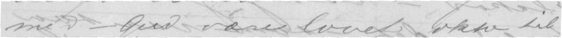med - Gud være lovet ikke til
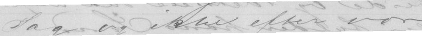Sag og ikke efter vor
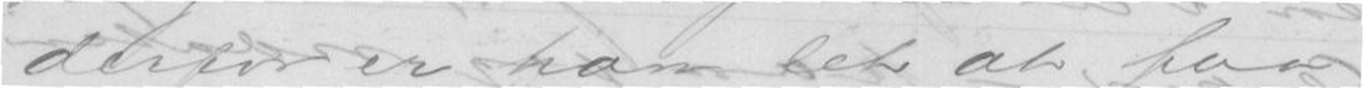derfor er han let at faa
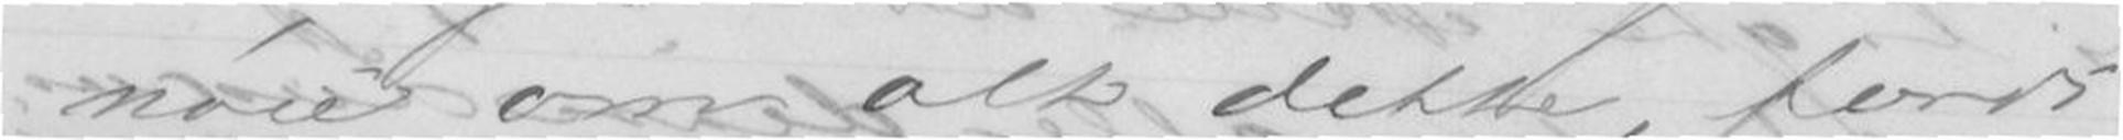nøie om alt dette, fordi
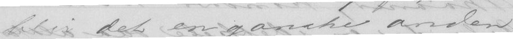blir det en ganske anden
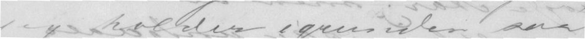jeg holder igrunden saa
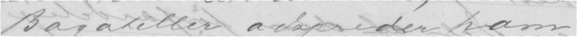Bagateller adspreder ham,
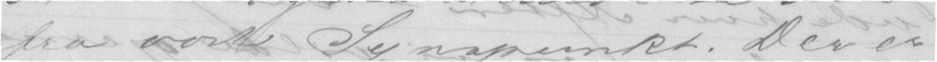fra vort Synspunkt. Der er
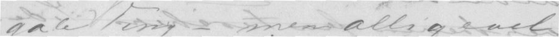gale Ting - men alligevel.
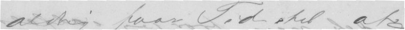aldrig faar Tid til at
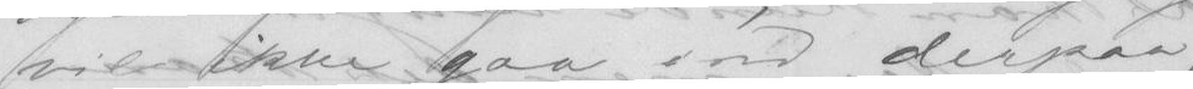vil ikke gaa ind derpaa,
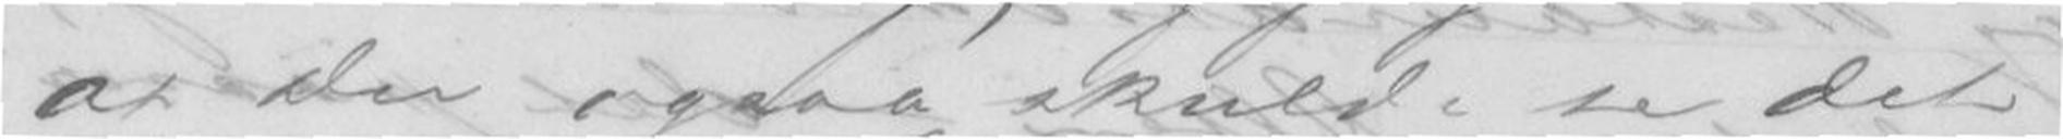at Du ogsaa skulde se det
